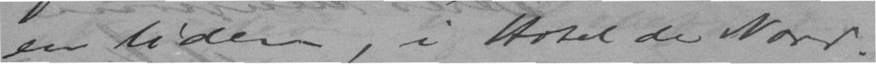en liden, i Hotel de Nord.
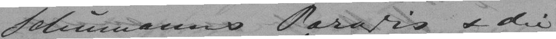Schumanns Paradis & die
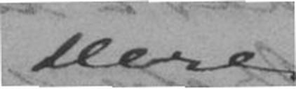Deres
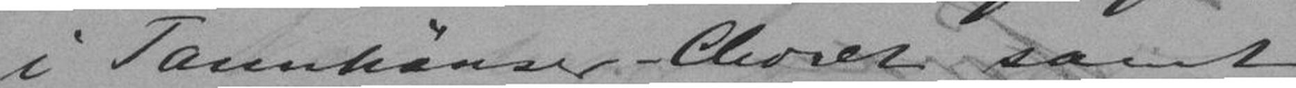i Tannhäuser-Choret samt
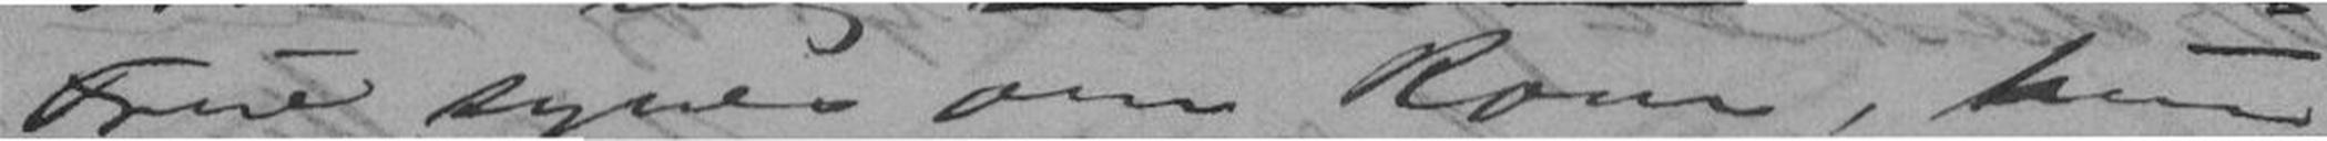Frue synes om Rom, hun
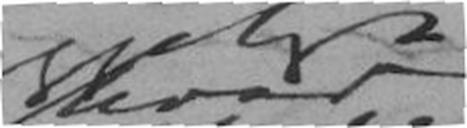hvad
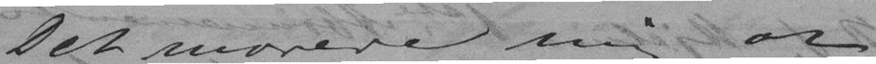Det morede mig at
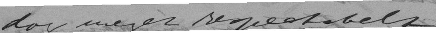dog meget respectabelt;
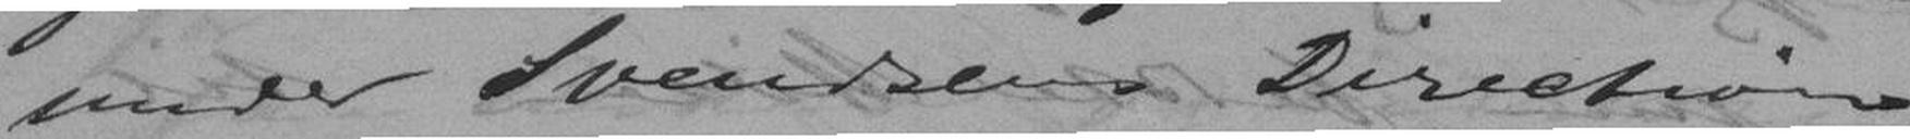under Svendsena Direktion,
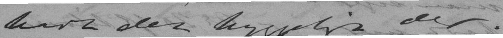havt det hyggeligt der.
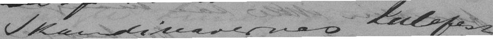Skandinavernes Julefest
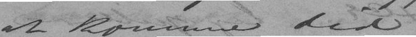at komme did
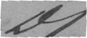DS.
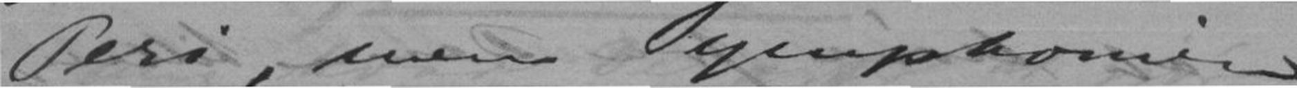Peri, men Symphonien
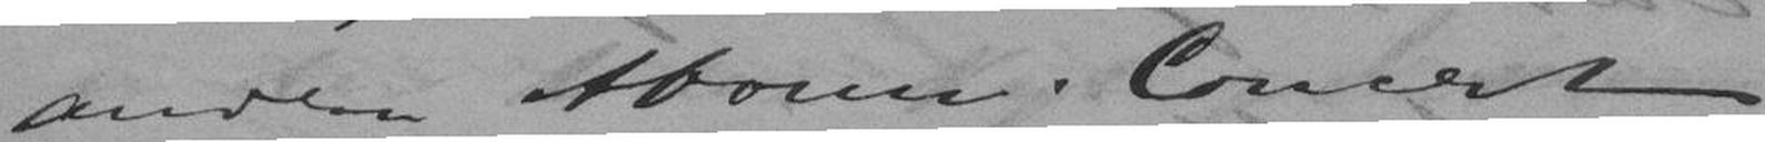anden Aboun. Concert
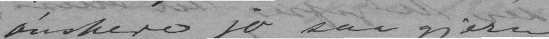ønskede jo saa gjerne
